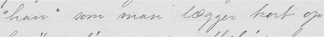"han" som man lægger kort op
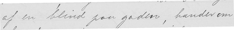af en blind paa gaden, handler om
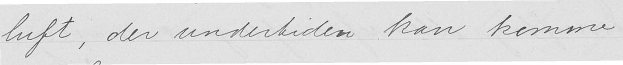luft, der undertiden kan komme
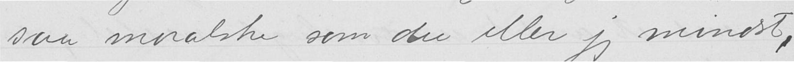saa moralske som du eller jeg mindst,
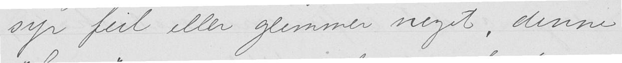syr feil eller glemmer noget, denne
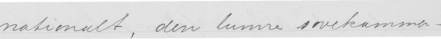nationalt, den lumre sovekammer-
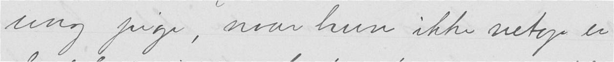ung pige, naar hun ikke netop er
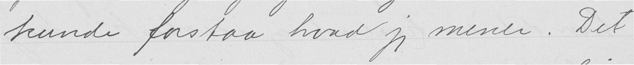kunde forstaa hvad jeg mener. Det
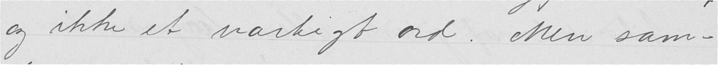og ikke et uartigt ord. Men sam-
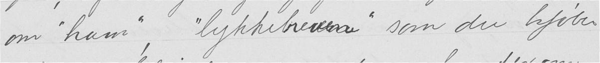om "ham", "lykkebrevene" som du kjøber
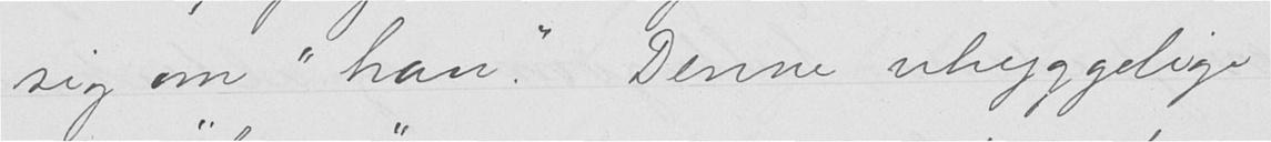sig om "han". Denne uhyggelige
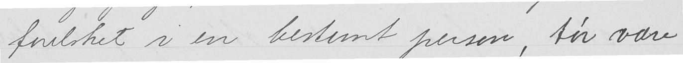forelsket i en bestemt person, tør være
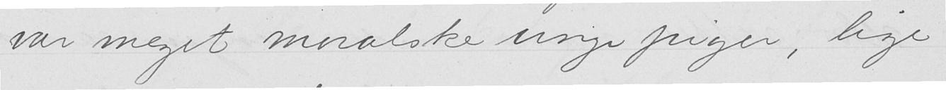var meget moralske unge piger, lige
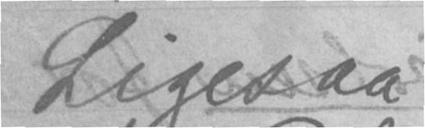Ligesaa:
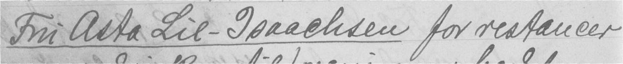Fru Asta Lil-Icaachsen for restancer
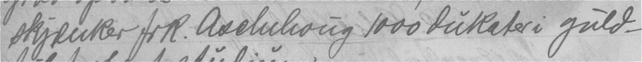skjænker frk. Aschehong 1000 dukater i guld -
